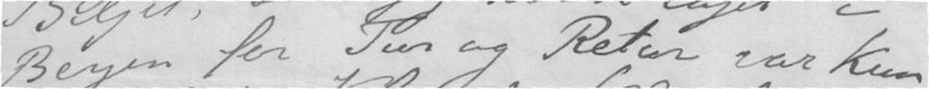Berjen for Tur Retur var kun
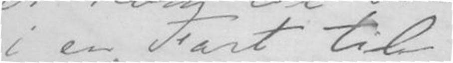i en Fart til
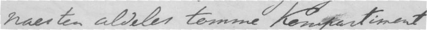næeste aldeles tomme Kompartiment
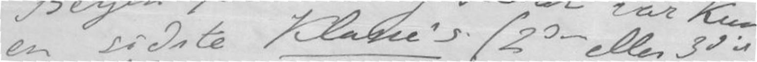en sidste Klasse's (2den eller 3die,
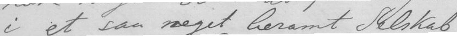i et saa meget berømt Selskab
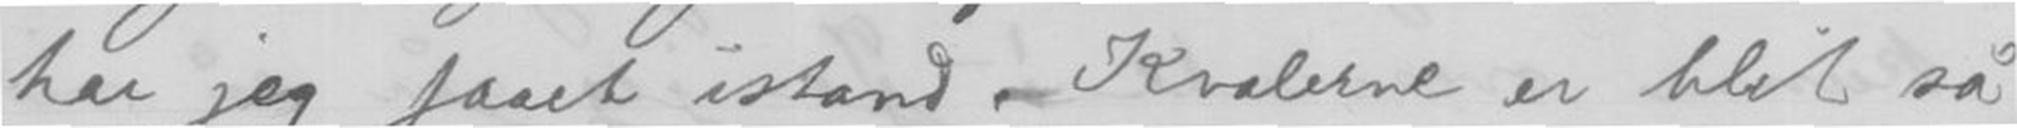har jeg faaet istand. Kvalerne er blit så
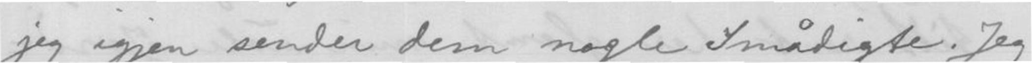jeg igjen sender dem nogle Smådigte. Jeg
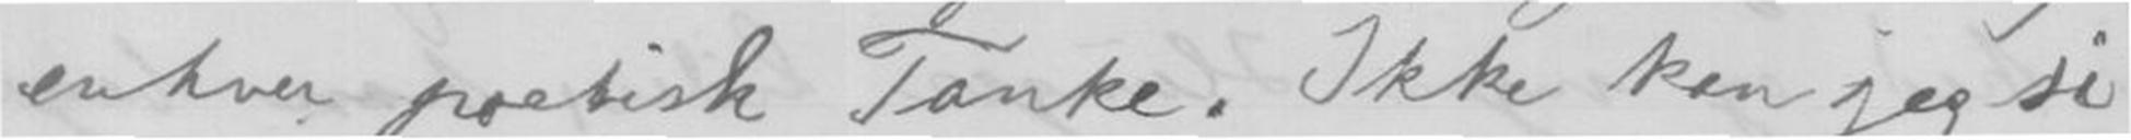enhver poetisk Tanke. Ikke kan jeg si
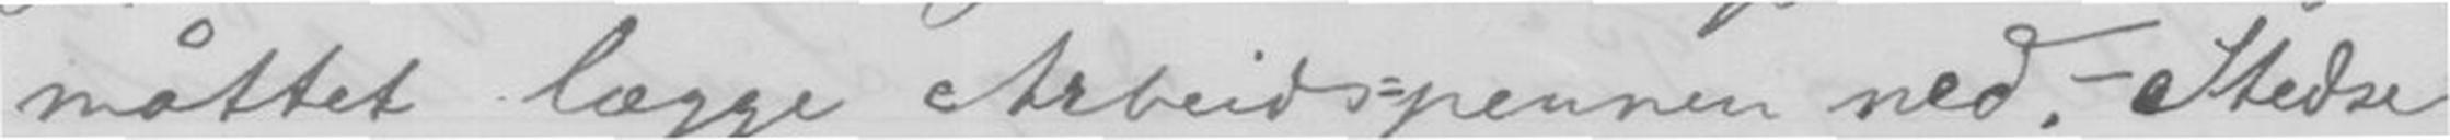måttet lægge Arbeis=pennen ned. - Stedse
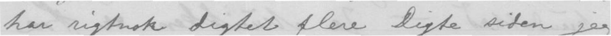har rigtignok digtet flere Digte siden jeg
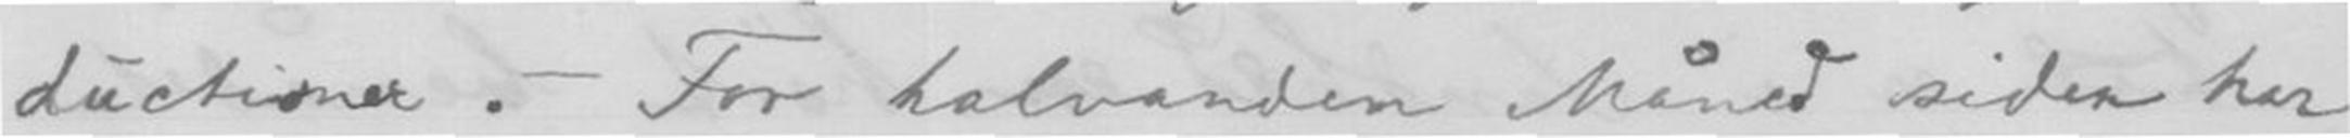ductioner. - For halvanden Måned siden har
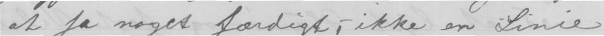at få noget færdigt, ikke en Linie
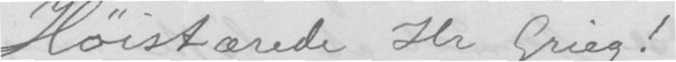Høistærede Hr Grieg!
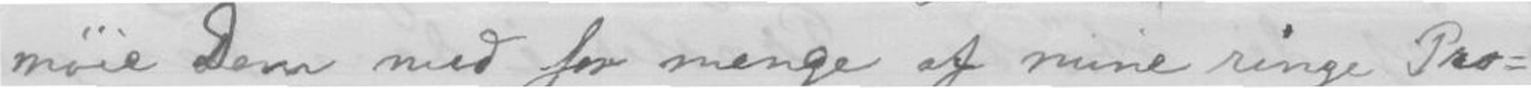møie Dem med for mange af mine ringe Pro-
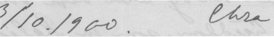3/10.1900Chra
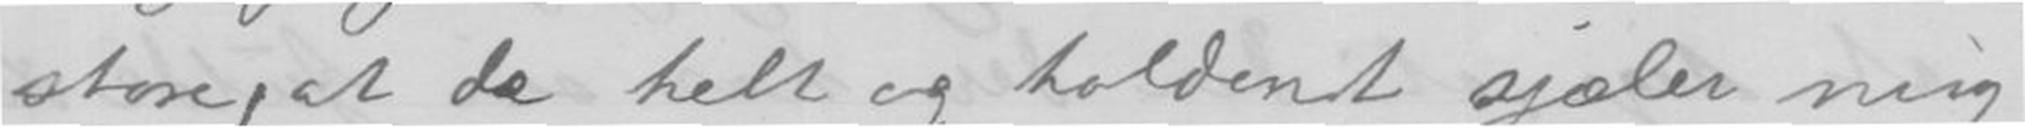store, at de helt og holdent sjæler mig
